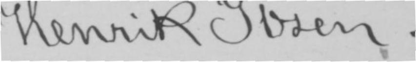Henrik Ibsen.
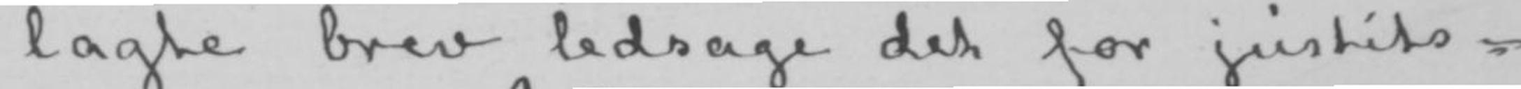lagte brev ledsage det for justits-
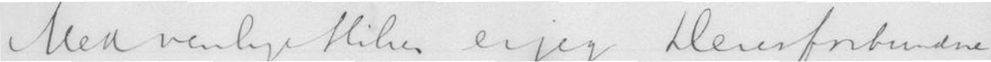Med vennlige Hilsen er jeg Deres forbundne
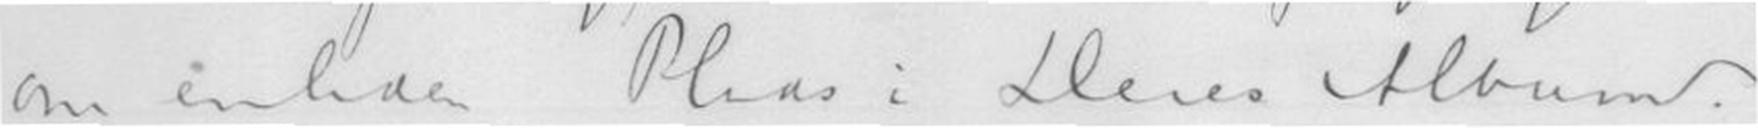om inlede Plads i Deres Album.
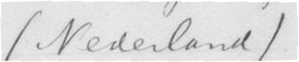(Nederland)
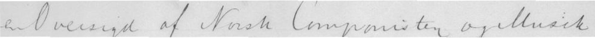en Översigt af Norsk Componister og Musik
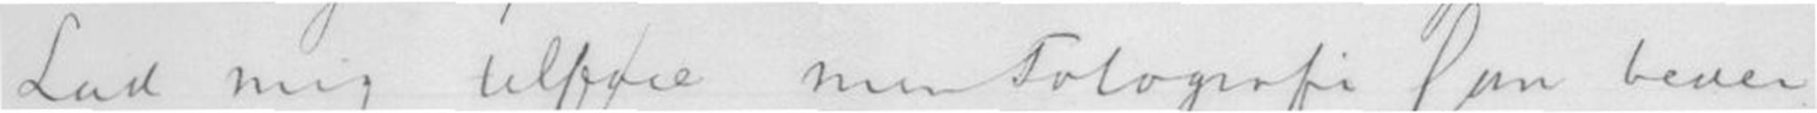Lad mig tilføre min Fotografi som beder
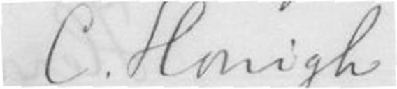C.Honigh
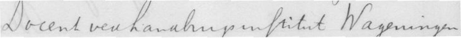Docent ved Landbrugsinstitut Wageningen
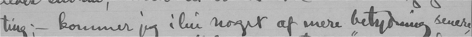ting; - kommer jeg ihu noget af mere bedydning senere
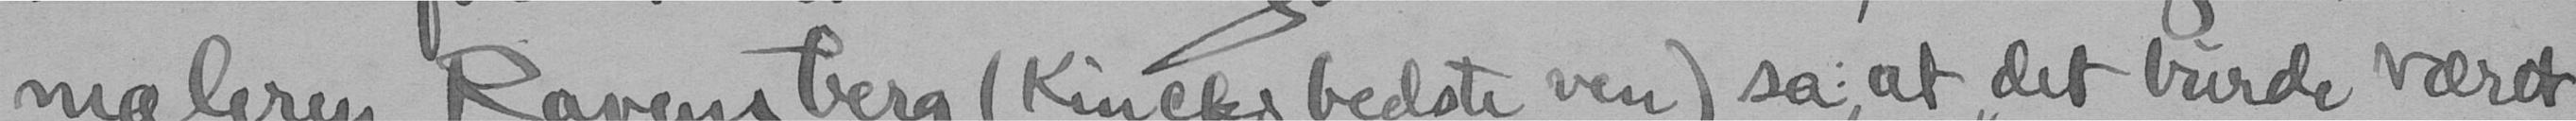maleren Ravensberg (Kincks bedste ven) sa, at det burde været
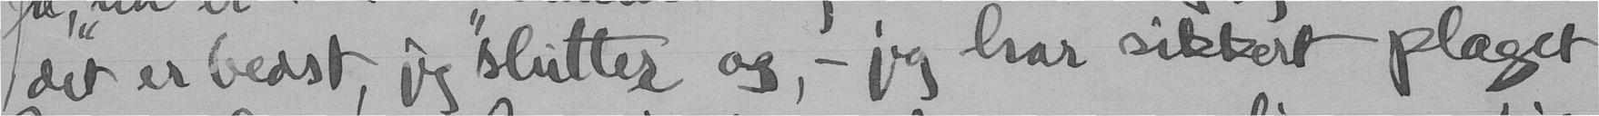det er bedst jeg slutter og, - jeg har sikkert plaget
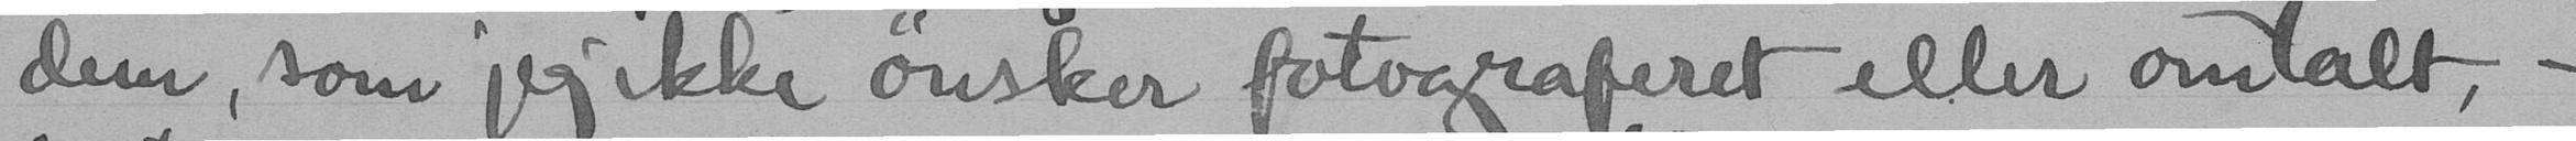dem, som jeg ikke ønsker fotograferet eller omtalt, -
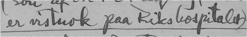er vistnok paa Rikshospitalet)
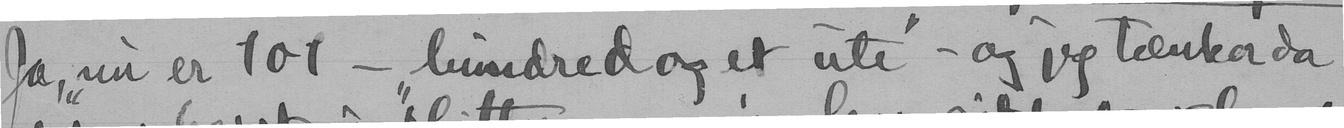Ja, "nu er 101 - "hundred og et ute" - og jeg tænker da
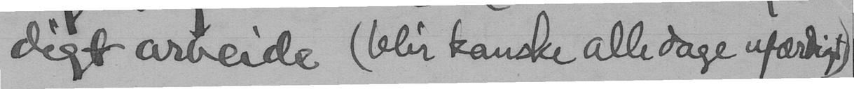digt arbeide (blir kanske alle dage ufærdigt.)
